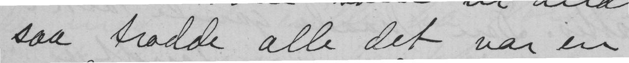saa trodde alle det var en
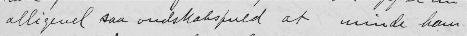alligevel saa ondskabsfuld at minde ham
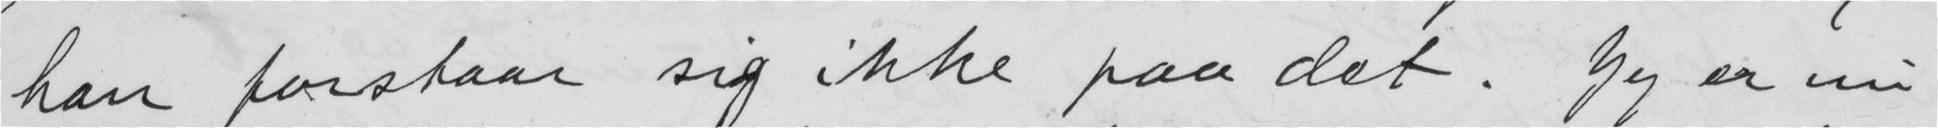han forstaar sig ikke paa det. Jeg er nu
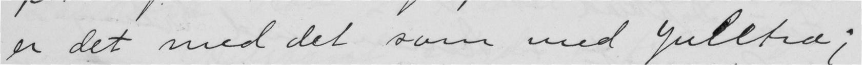er det med det som med Juletræ;
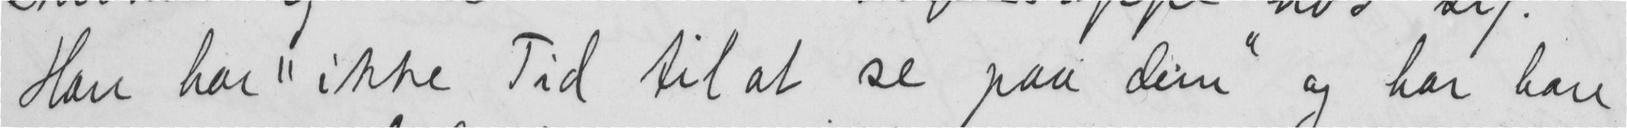Han har "ikke Tid til at se paa dem" og har han
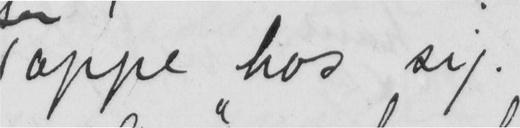oppe hos sig.
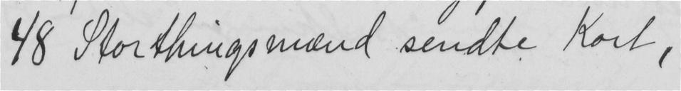28 Storthingsmænd sendte Kort,
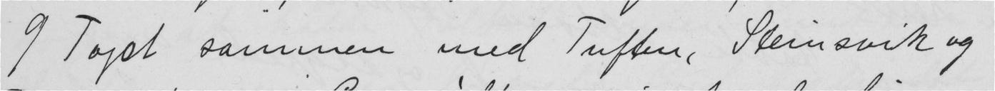9 Toget sammen med Tuften, Steinsvik og
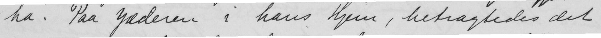ha. Paa Jæderen i hans Hjem, betragtedes det
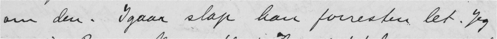om den. Igaar slap han forresten let. Jeg
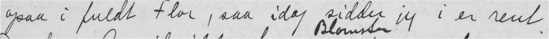ogsaa i fuldt Flor, saa idag sidder jeg i er rent
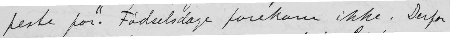feste for". Fødselsdage forekom ikke. Derfor
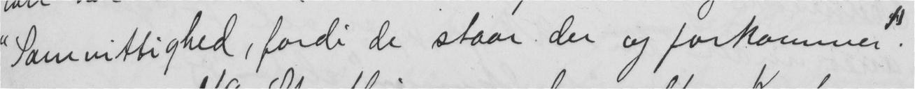"Samvittighed, fordi de staar der og forkommer".
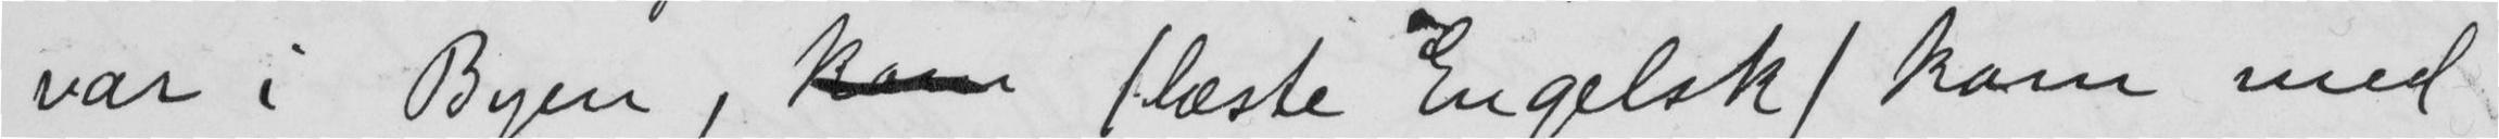var i Byen,  (læste Engelsk) kom med
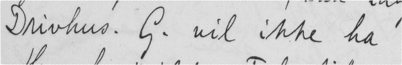Drivhus. G. vil ikke ha
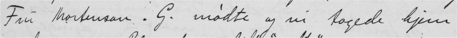Fru Mortenson. G. mødte og vi tagede hjem
